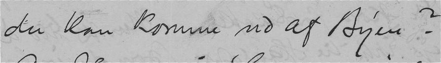du kan komme ud af Byen?
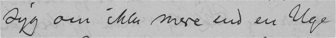syg om ikke mere end en Uge
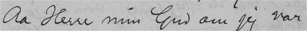Aa Herre min Gud om jeg var
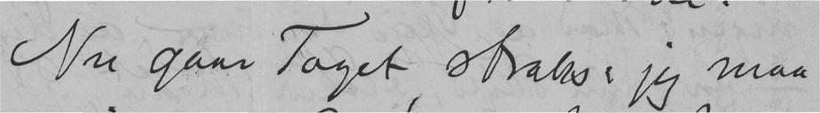Nu gaar Toget straks, jeg maa
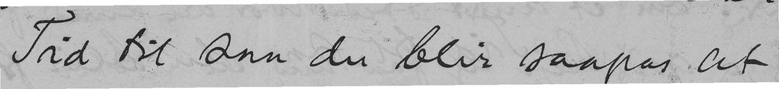Tid til saa du blir saapas at
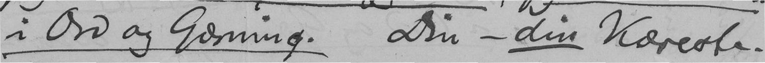i Ord og Gærning. Din - din Kæreste.
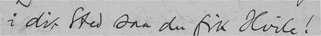i dit Sted saa du fik Hvile!
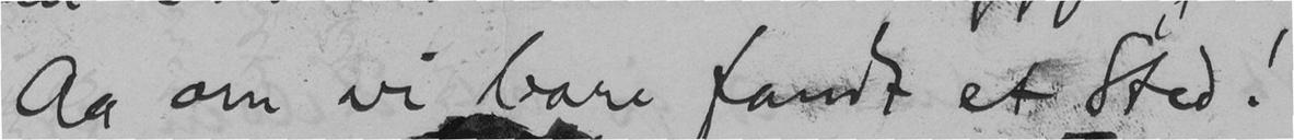Aa om vi bare fandt et Sted!
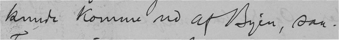kunde komme ud af Byen, saa.
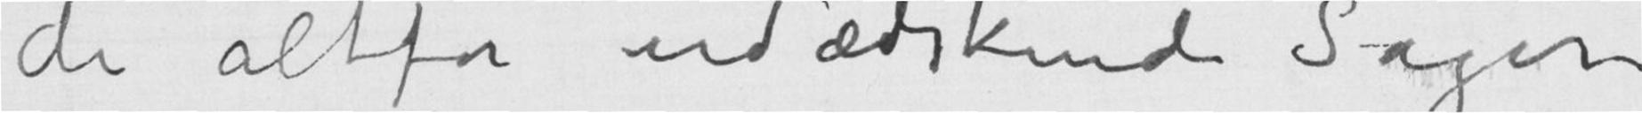de altfor udædskende Sager
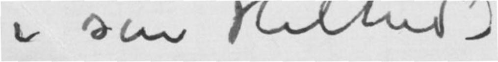i sin Helhed)
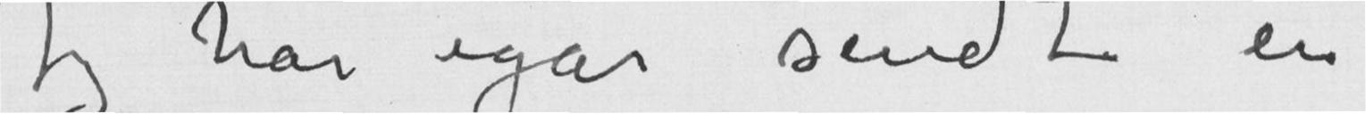Jeg har igår sendt en
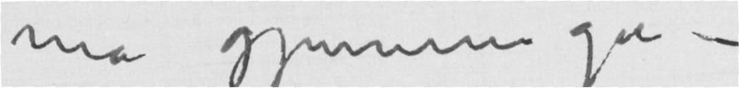må gjennemgå –
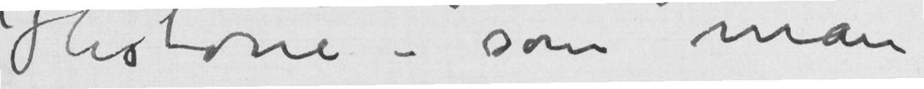Historie – som man
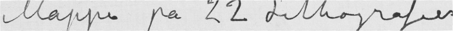Mappe på 22 Lithografier
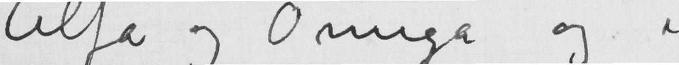Alfa og Omega og i
